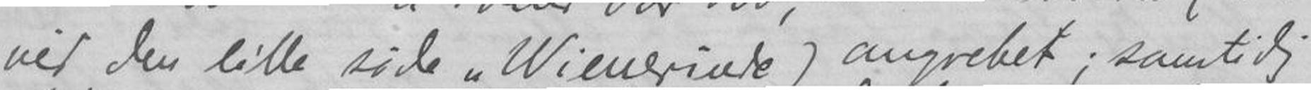ved den lille søde "Wienerinde) angrebet; samtidig
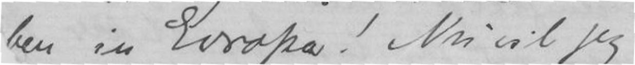ben in Europa! Nu vil jeg
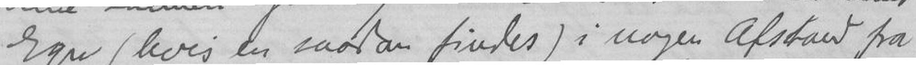Egre (hvis en saadan findes) i nogen Afstand fra
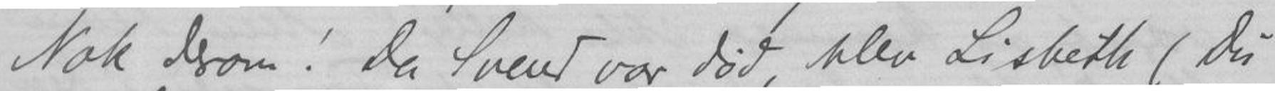Nok derom! Da Svend var død, blev Lisbeth (Du
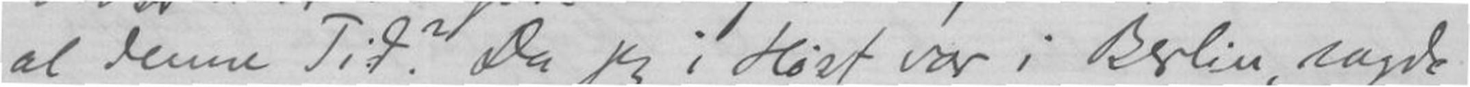al denne Tid? Da jeg i Høst var i Berlin, sagde
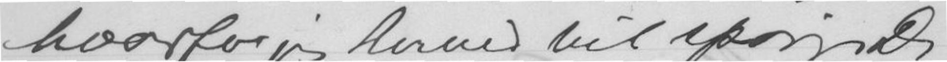hvorfor jeg dermed vil spørge Dig
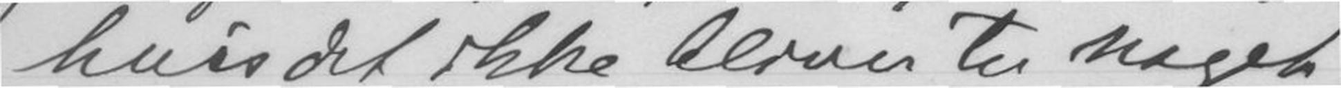hvis det ikke bliver til noget,
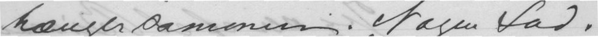hænger sammen. Nogen Ind-
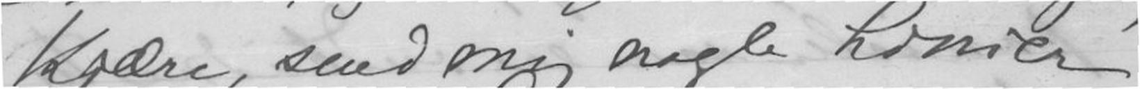Kjære, send mig nogle Linier
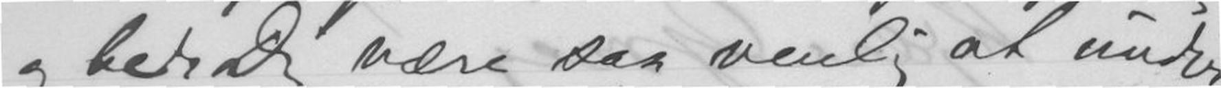og bede Dig være saa venlig at under-
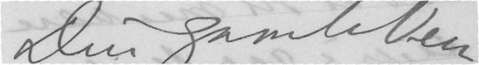Din gamle Ven
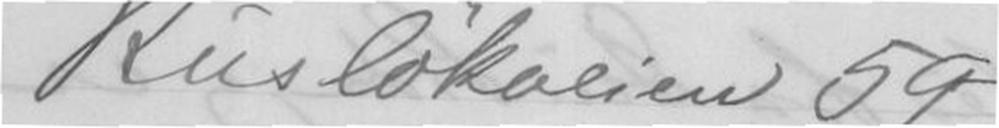Rusløkveien 59
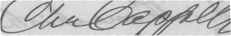Chr Cappelen
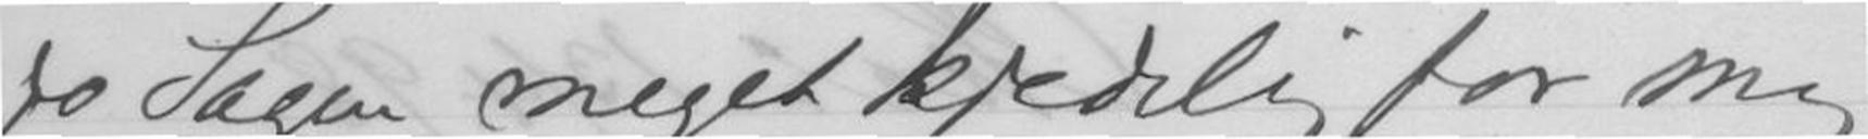jo sagen meget kjedelig for mig
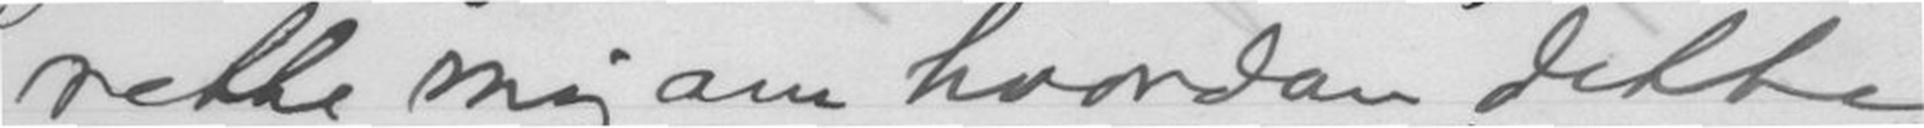rette mig om hvordan dette
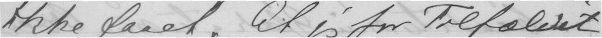ikke faaet. At jeg for Tilfældet
